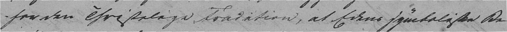for den christelige Tradition, at Edens symboliske Be-
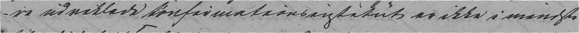re udviklede Confirmationsinstitut er ikke i mindste
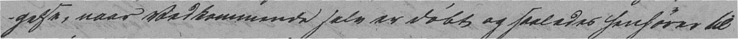gelse, naar Vedkommende selv er døbt og saaledes henhører til
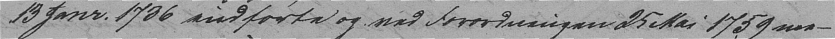13 Janr. 1736 indførte og ved Forordningen 25 Mai 1759 me-
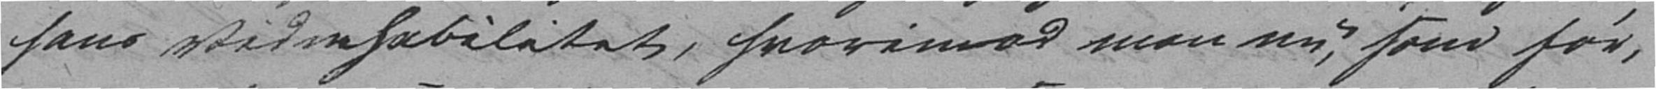hans Vidnehabilitet, hvorimod man nu, som før,
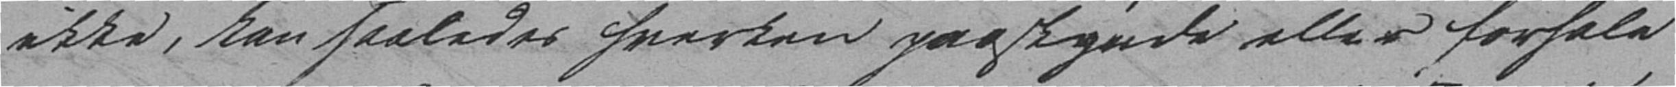ikke, kan saaledes hverken paaskynde eller forhale
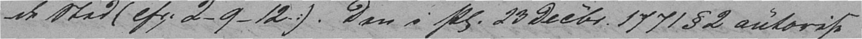de Sted (cfr. 2-9-12. :). Den i Pl. 23 Decbr. 1771 § 2 autorise-
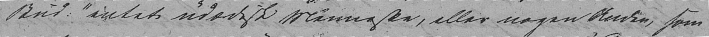Bud: "intet udædisk Menneske, eller nogen Anden, som
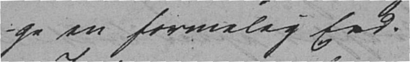ge en formelig Eed.
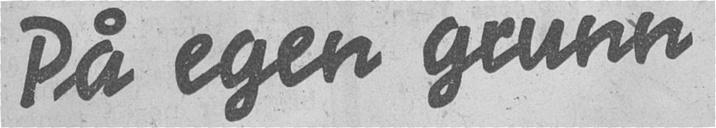På egen grunn
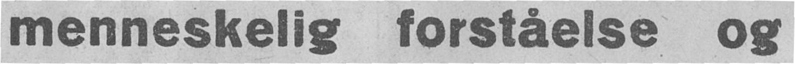menneskelig forståelse og
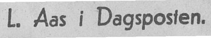L. Aas i Dagsposten.
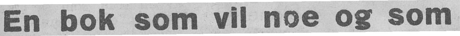En bok som vil noe og som
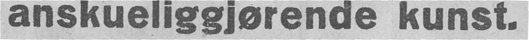anskueliggjørende kunst.
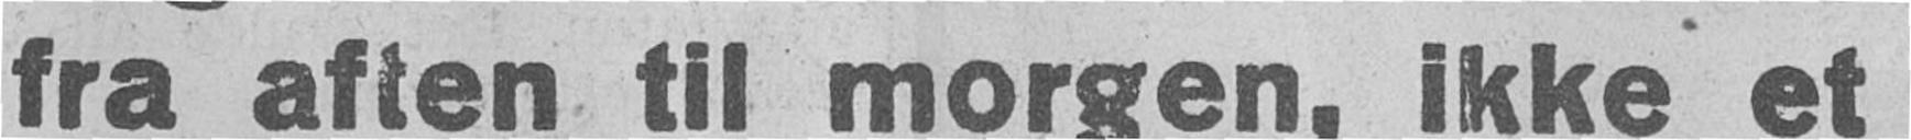fra aften til morgen, ikke et
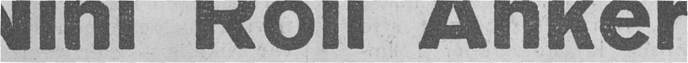Nini Roll Anker
